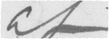af
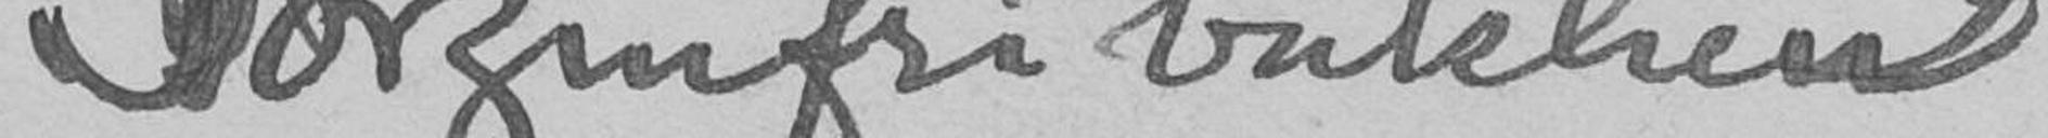Sorgenfribakken
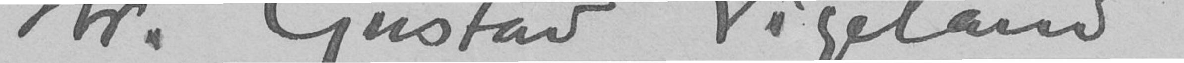Hr. Gustav Vigeland
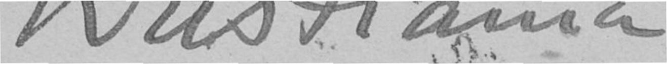Kristiania
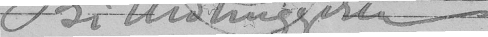Billedhuggeren
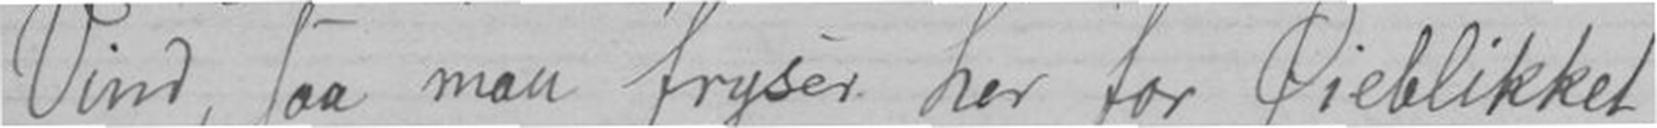Vind, saa man fryser her for Øieblikket,
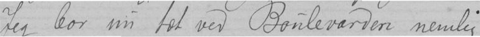Jeg bor nu tæt ved Boulevarden nemlig
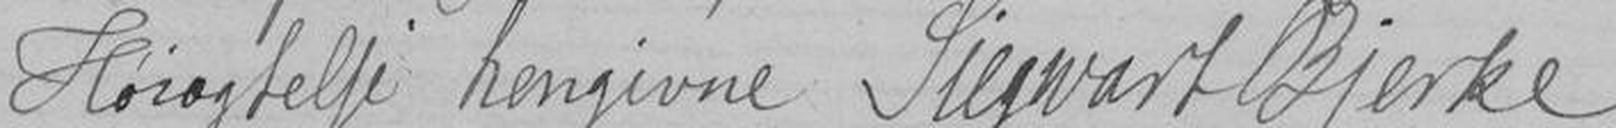Høiagtelse hengivne Siegwart Bjerke
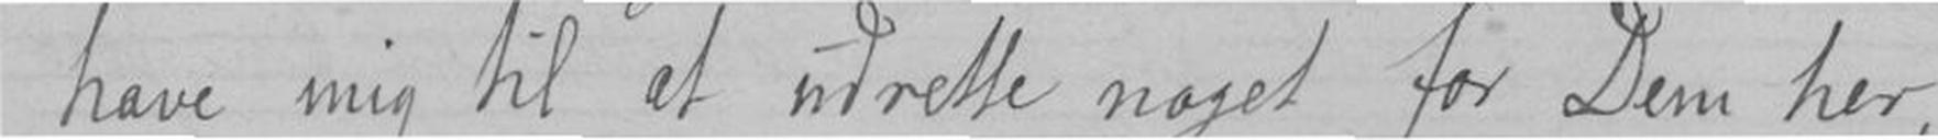have mig til at udrette noget for Dem her,
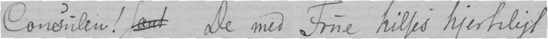Consulen! sant De med Frue hilses hjerteligt
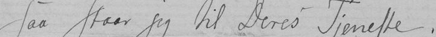saa staar jeg til Deres Tjeneste.
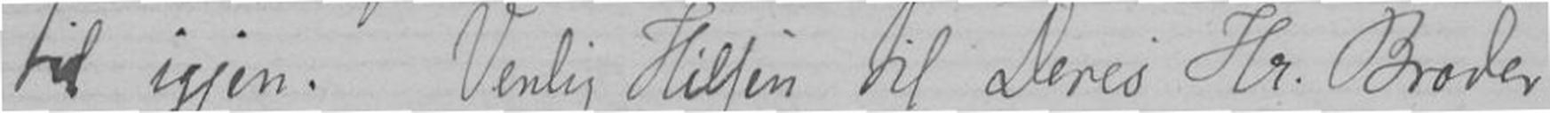til igjen. Venlig Hilsen til Deres Hr. Broder
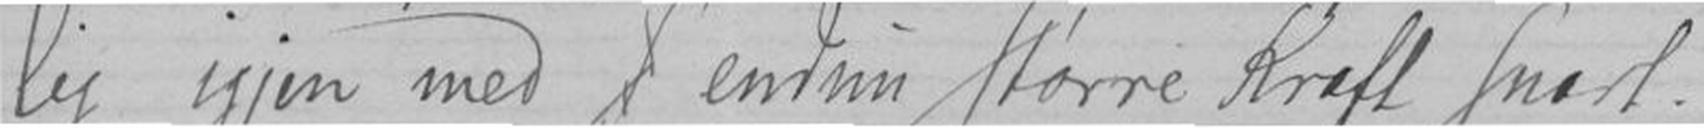lig igjen med endnu større Kraft snart.
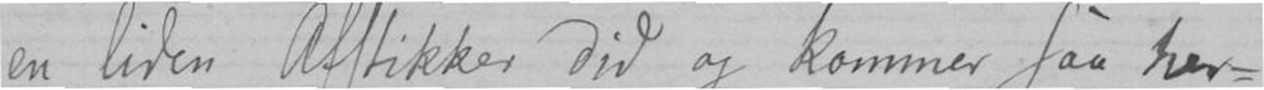en liden Afstikker did og kommer saa her-
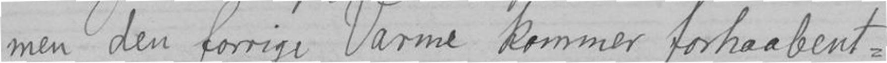men den forrige Varme kommer forhaabent-
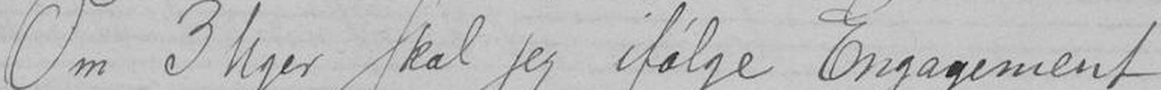Om 3 Uger skal jeg ifølge Engagement
